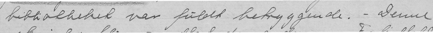bibliotheket var fuldt betryggende. - Denne
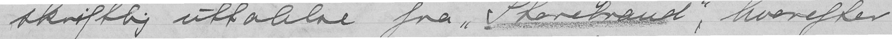skriftlig uttalelse fra "Storebrand", hvorefter
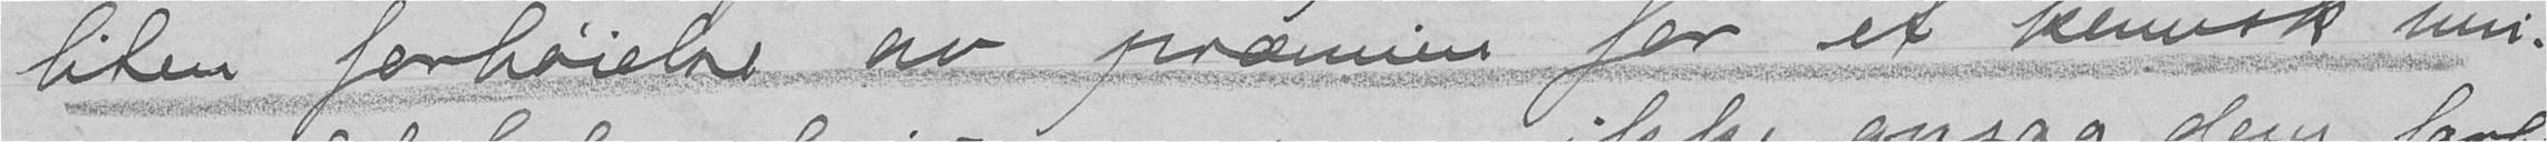liten forhøielse av. præmien for et kemisk uni-
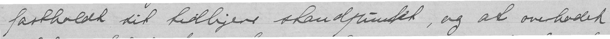fastholdt sit tidligere standpunkt, og at overhodet
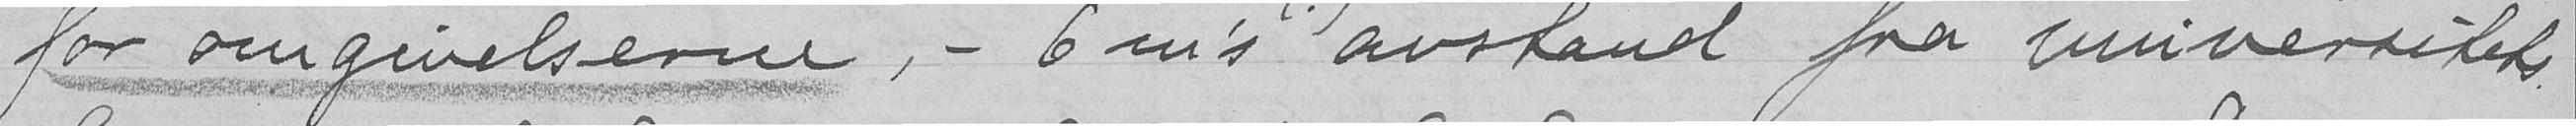for omgivelserne, - 6 m's(?) avstand fra universitets
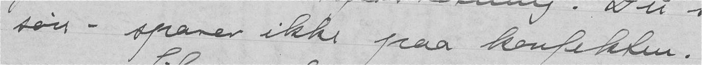søn - sparer ikke paa konfekten.
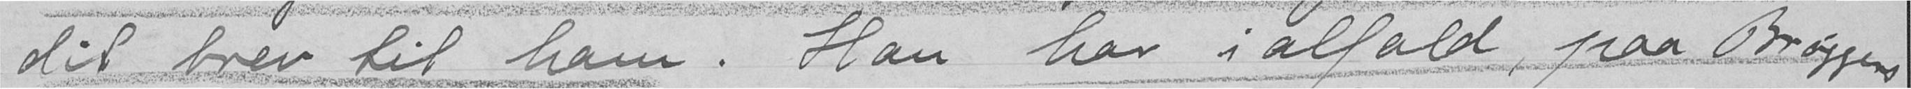dit brev til ham. Han har ialfald, paa Brøggers
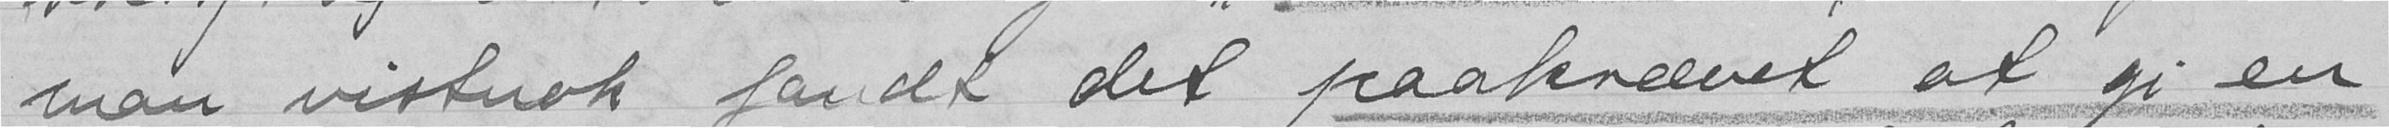man vistnok fandt det paakrævet at gi en
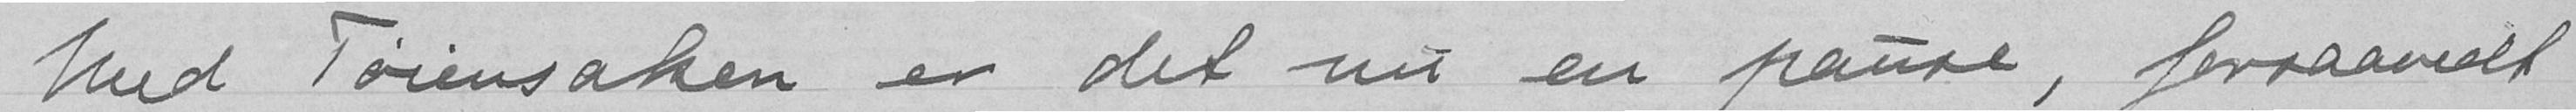Med Tøiensaken er det nu en pause, forsaavidt
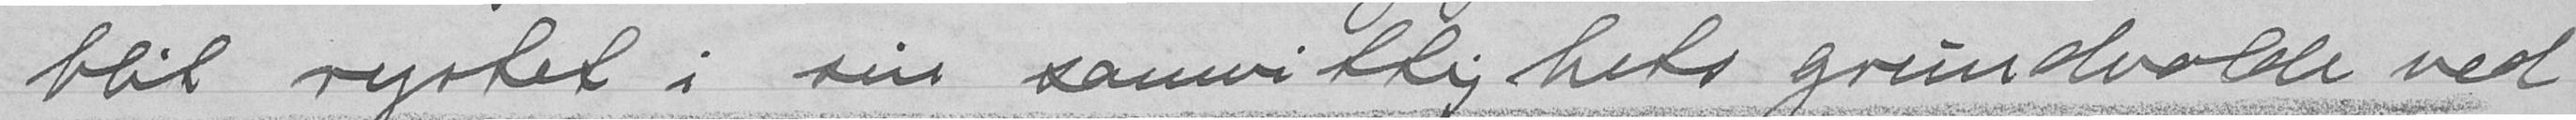blit rystet i sin samvitlighets grundvolde ved
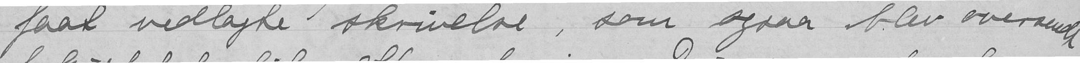faat vedlagte skrivelse, som ogsaa blev oversendt
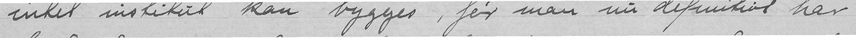intet institut kan bygges, før man nu definitivt har
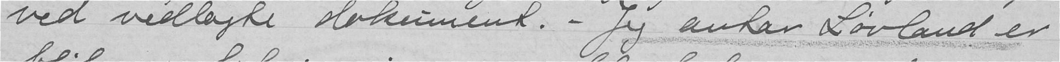ved vedlagte dokument.- Jeg antar Løvland er
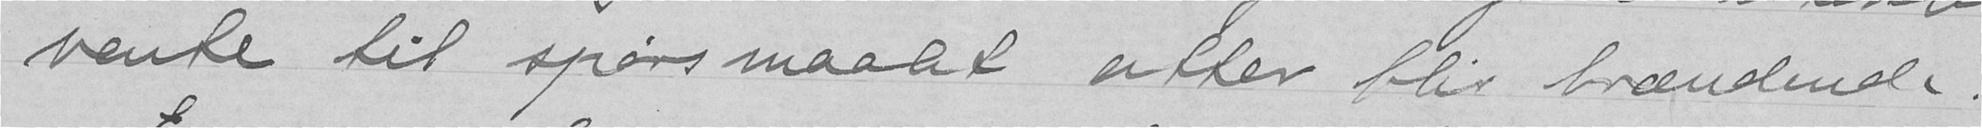vente til spørsmaalet efter blir brændende.
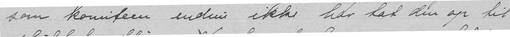som komiteen endnu ikke har tat den op til
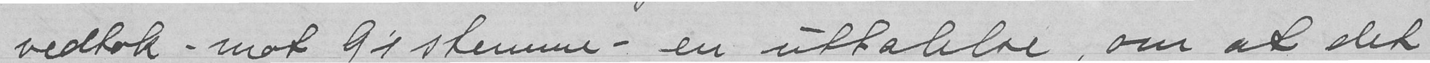vedtak - mot G's stemme - en uttalelse, om at det
